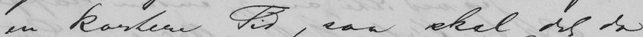en kortere Tid, saa skal det da
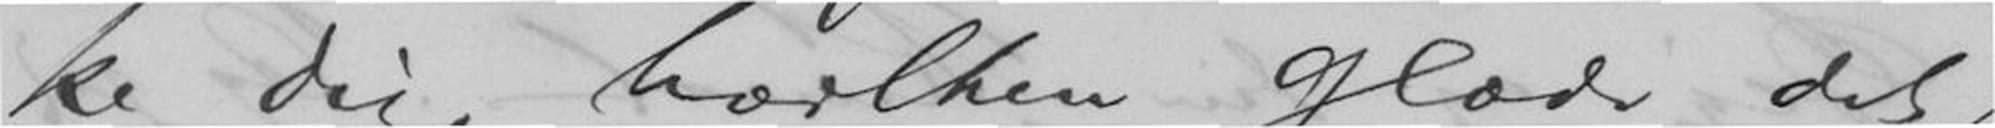ke dig, hvilken Glæde det
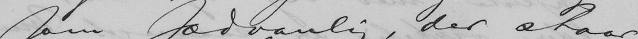som sædvanlig, der staar
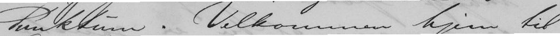Punktum. Velkommen hjem til
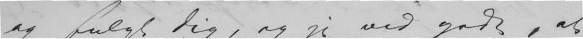og fulgt dig, og jeg ved godt,
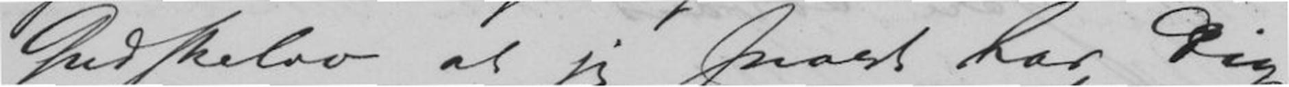Gudskelov at jeg snart har Dig
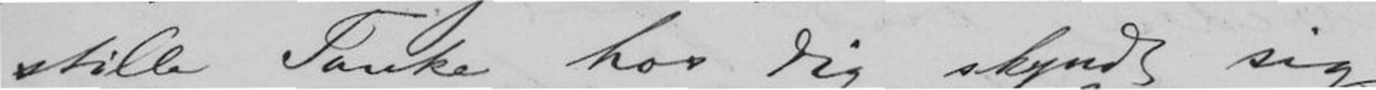stille Tanke hos dig skyndt sig
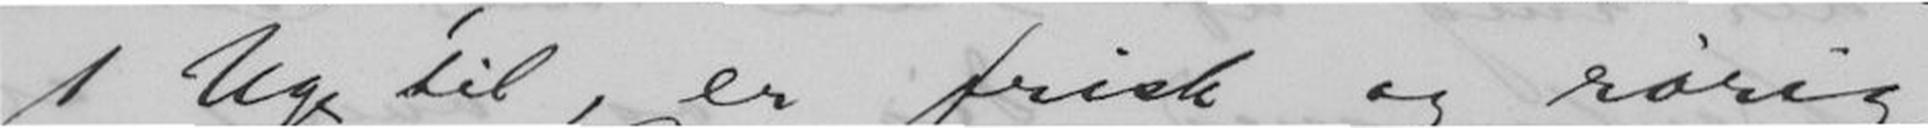1 Uge til, er frisk og rørig
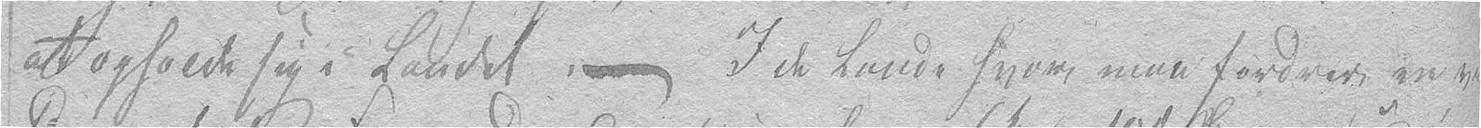at opholde sig i Landet. – I de Lande hvor man fordrer en vis
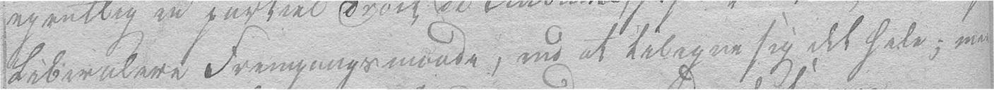liberalere Fremgangsmaade, end at tilegne sig det hele; men
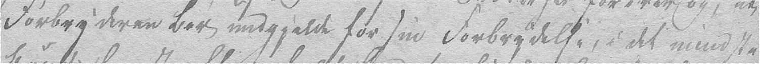Forbryderen bør undgjælde for sin Forbrydelse, i det mindste
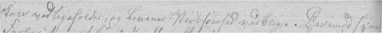kan vedligeholdes, og Lovenes Virksomhed vedblive. Derimod synes
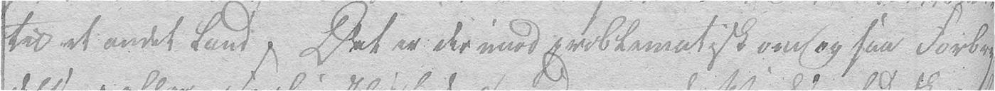til et andet Land. Det er derimod problematisk om ogsaa Forbry-
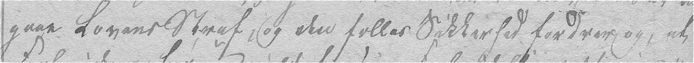gaae Lovens Straf, og den fælles Sikkerhed fordrer og, at
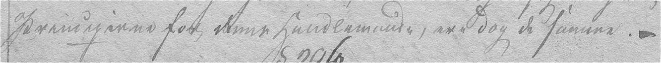Principerne for denne Handlemaade, ere dog de samme. –
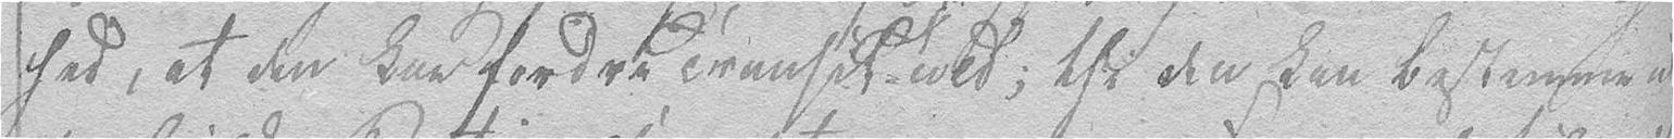hed, at den kan fordre grændse-Told; thi den kan bestemme un-
